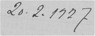20.2.1927
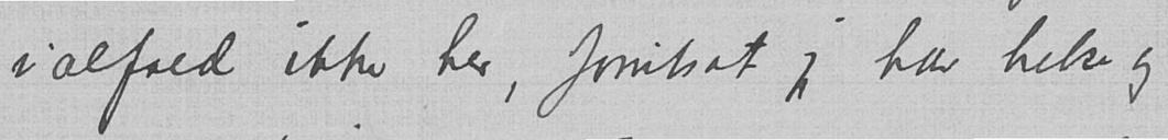ialfald ikke her, forutsat jeg har helse og
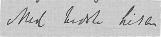Med bedste hilsen
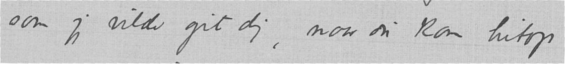som jeg vilde git dig, naar du kom hitop
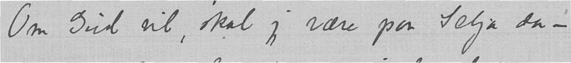Om Gud vil, skal jeg være paa Selja da -
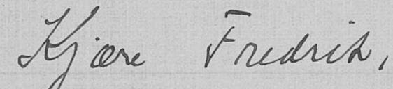Kjære Fredrik,
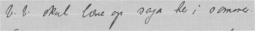b.b. skal læse op saga her i sommer.
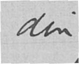din
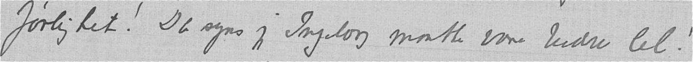førlighet. Da syns jeg Ingeborg maatte være bedre lel!
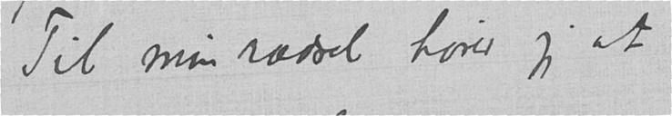Til min rædsel hører jeg at
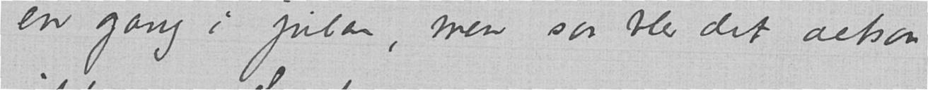en gang i julen, men saa blev det altsaa
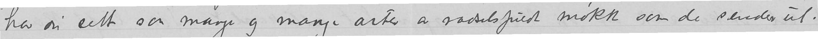har du sett saa mange og mange arter av rædselsfuldt møkk som de sender ut.
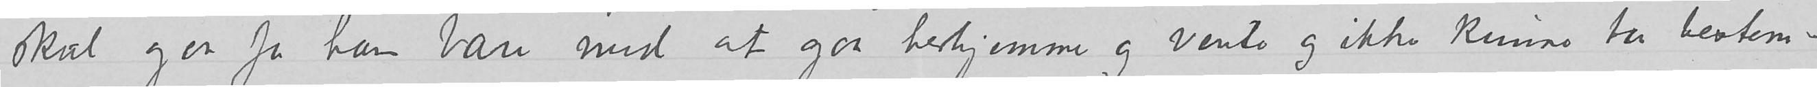skal gaa for han bare med at gaa herhjemme og vente og ikke kunne ta bestem-
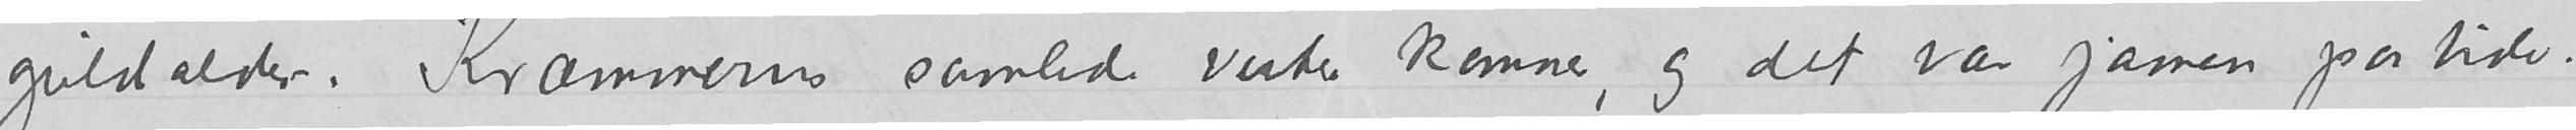guldalder. Krammerns samlede verker kommer, og det var jamen paa tide.
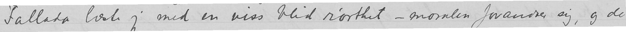Fallada læste jeg med en viss blid rørthet – moralen forandrer sig, og de
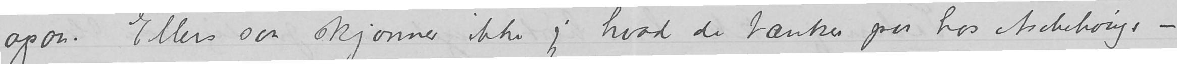ogsaa.  Ellers saa skjønner ikke jeg hvad de tænker paa hos Aschehougs –
